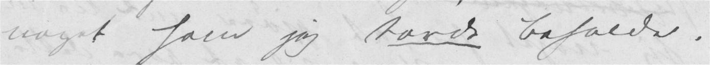noget som jeg torde beholde.
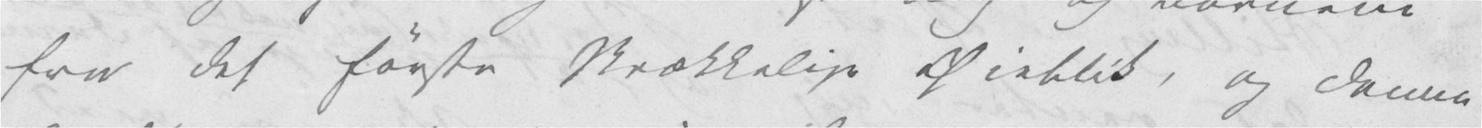fra det første skrækkelige Øieblik, og denne
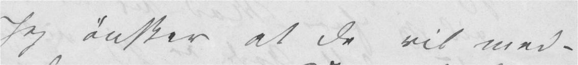Jeg ønsker at De vil med-
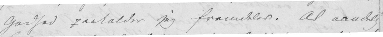Godhed paakalder jeg fremdeles. Al aandelig
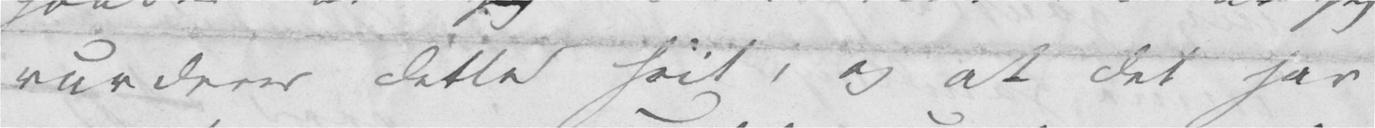vurderer dette høit, og at det har
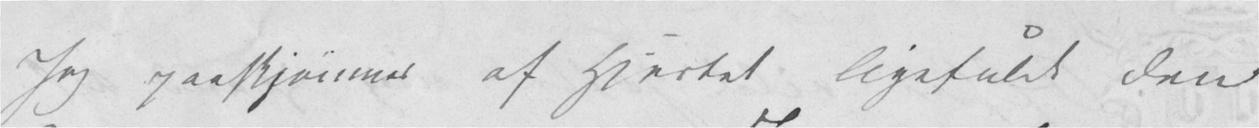Jeg paaskjønner af Hjertet ligefuldt den
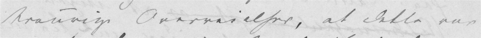traurige Overveielser, at dette var
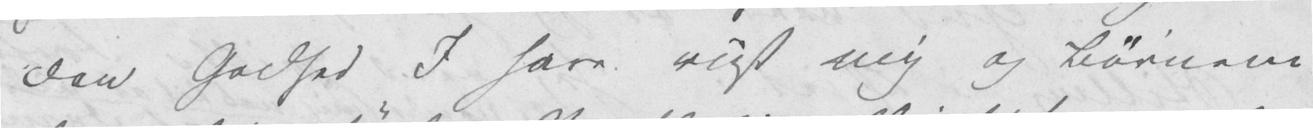den Godhed I have viist mig og Børnene
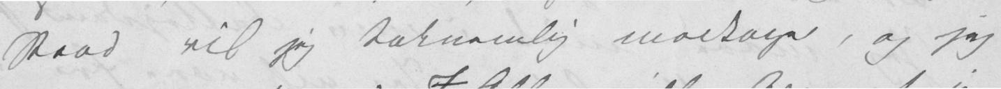Raad vil jeg taknemlig modtage, og jeg
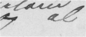og al
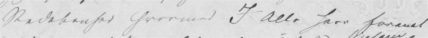Redebonhed hvormed I Alle have forenet
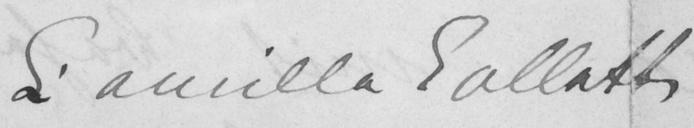Camilla Collett
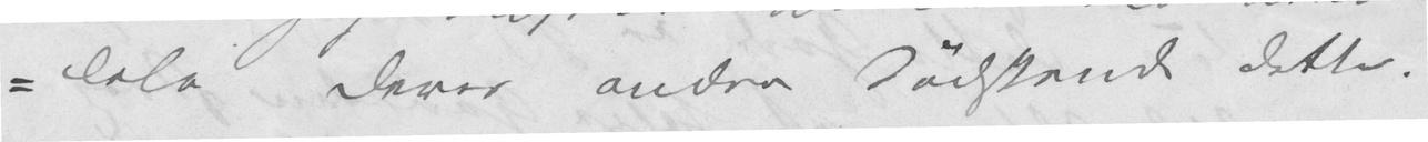dele Deres andre Sødskende dette.
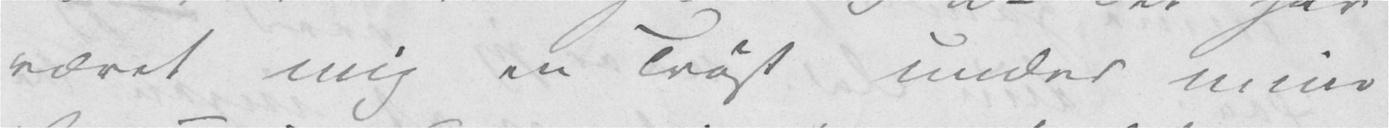været mig en Trøst under mine
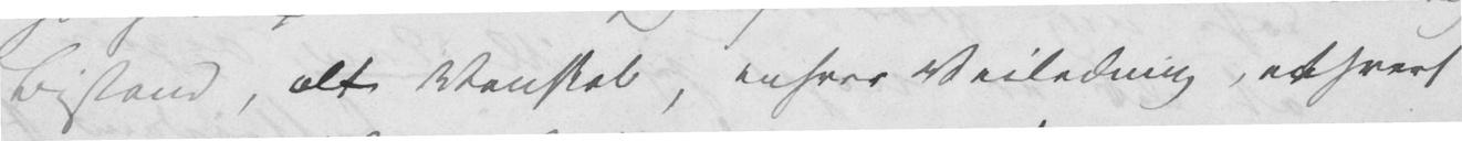Bistand, alt Venskab, enhver Veiledning, ethvert
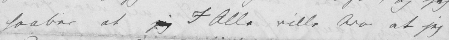haaber at jeg I Alle ville tro at jeg
